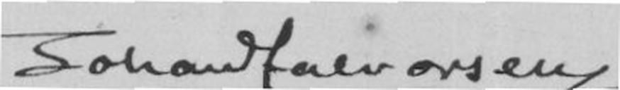Johan Halvorsen
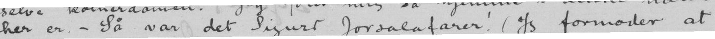her er. - Så var det Sigurd Jorsalafarer! Jeg formoder at
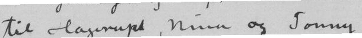til Hagerups, Nina og Tonny.
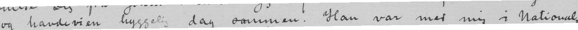og havde vi en hyggelig dag sammen. Han var med mig i National-
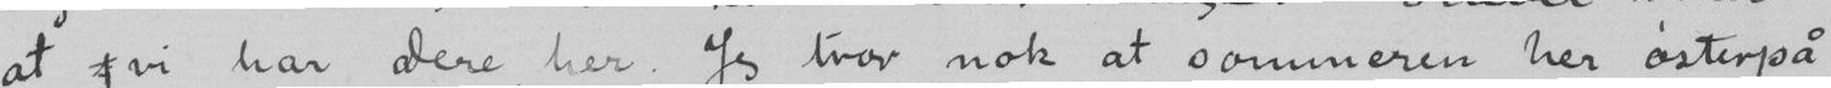at vi har dere her. Jeg tror nok at sommeren her østerpå
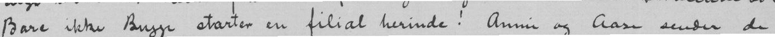Bare ikke Bugge starter en filial herinde! Annie og Aase sender de
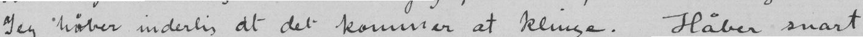Jeg håber inderlig at det kommer at klinge. Håber snart
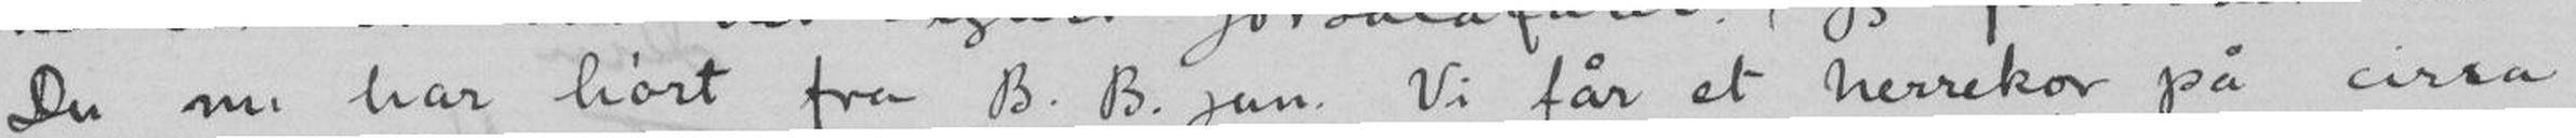Du nu har hørt fra B.B.jun. Vi får et herrekor på circa
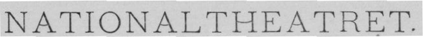NATIONALTHEATRET
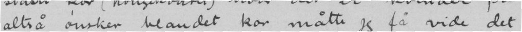altså ønsker blandet kor måtte jeg få vide det
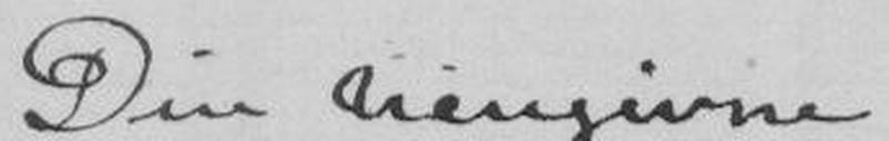Din hengivne
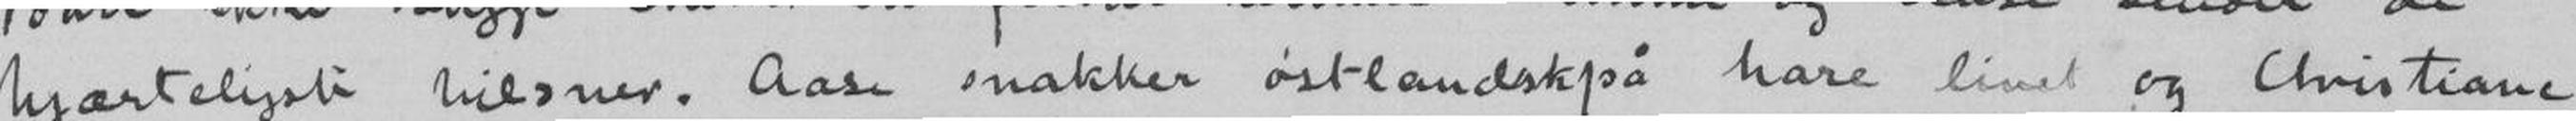hjæteligste hilsner. Aase snakker østlandsk på hare livet og Christiane
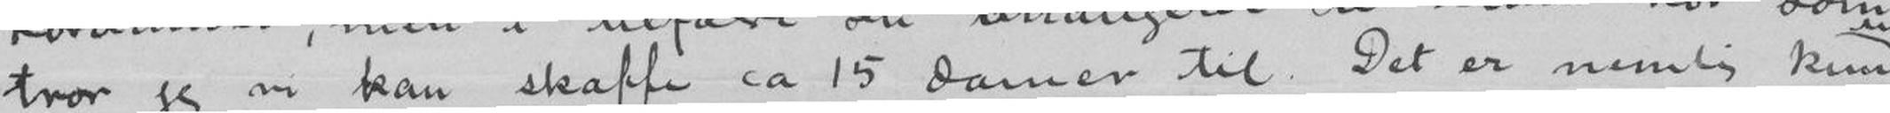tror jeg vi kan skaffe ca 15 damer til. Det er nemlig ku
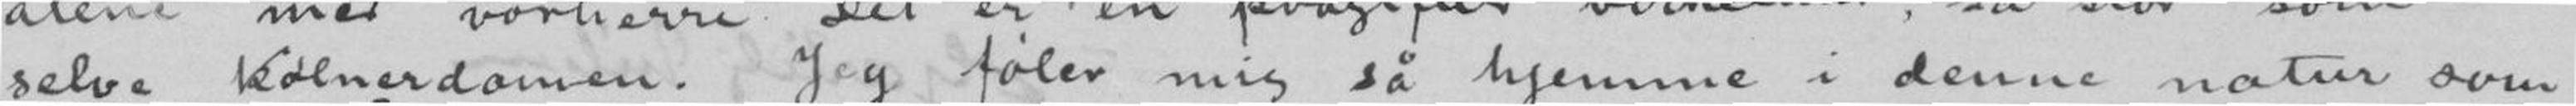selve Kølnerdomen. Jeg føler mig så hjemme i denne natur som
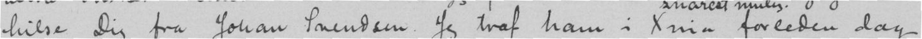hilse Dig fra Johan Svendsen. Jeg traf ham i Xnia forleden dag
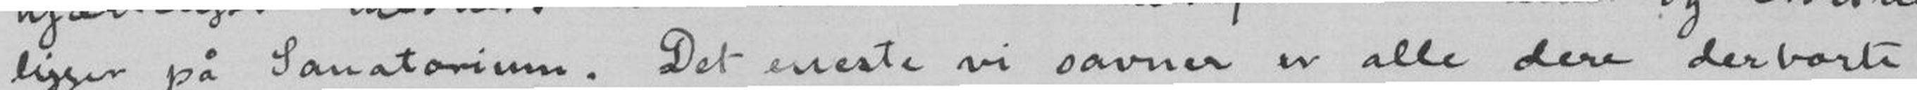ligger på Sanatorium. Det eneste vi savner er alle dere derborte
